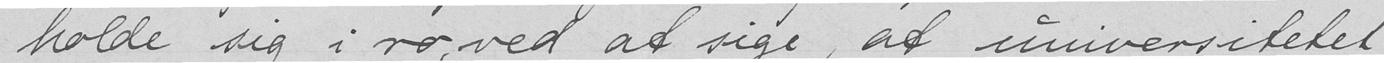holde sig i ro , ved at sige, at universitetet
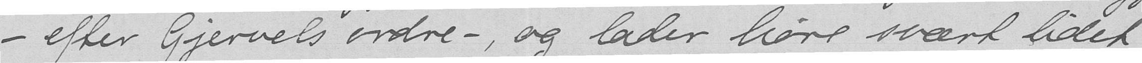- efter Gjervels ordre -, og lader høre svært lidet
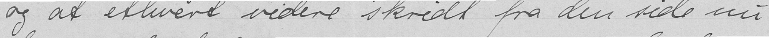og at ethvert videre skridt fra den side nu
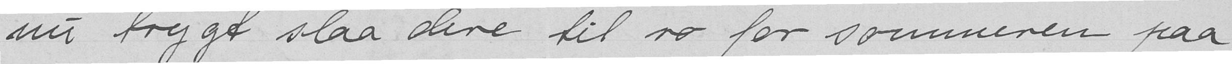nu trygt slaa dere til ro for sommeren paa
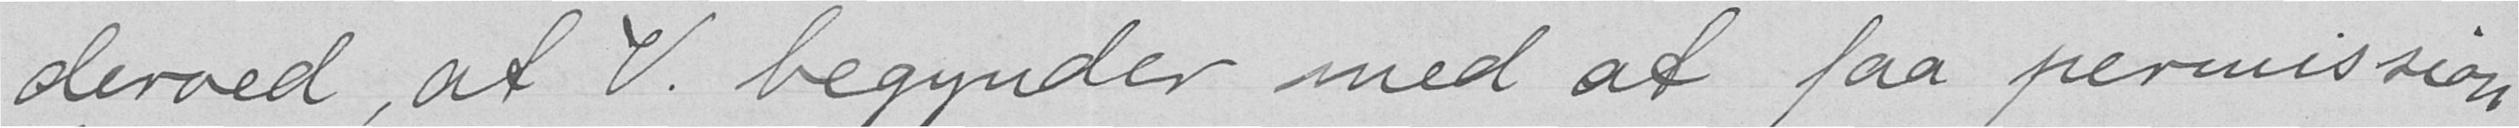derved, at V. begynder med at faa permission
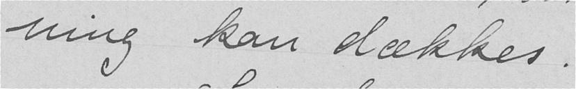ning kan dækkes.
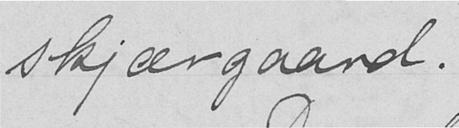skjærgaard.
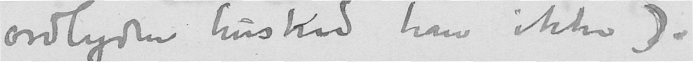ordlyden husked han ikke).
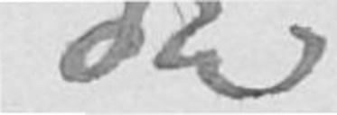da
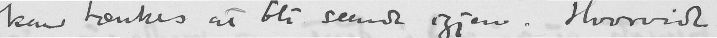kan tænkes at bli seende igjen. Hvorvidt
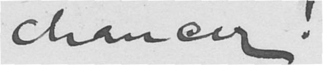chancer!
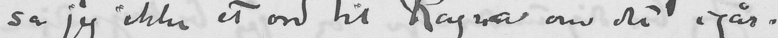sa jeg ikke et ord til Ragna om det igår.
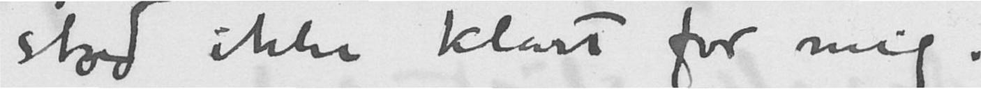stod ikke klart for mig.
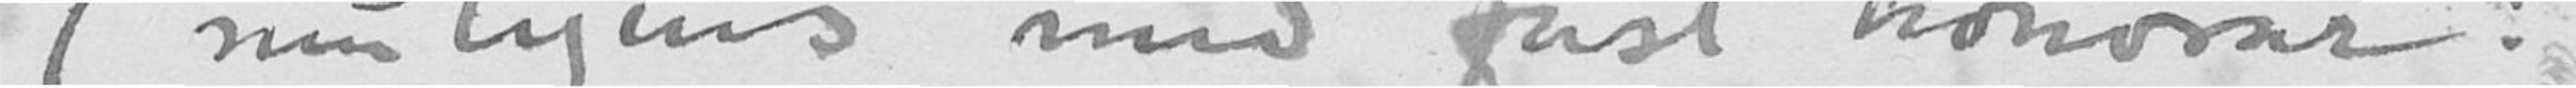(muligens med fast honorar?
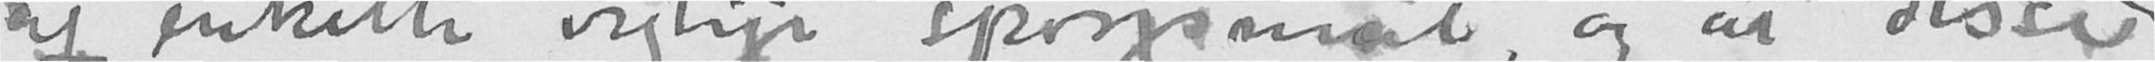af enkelte vigtige spørgsmål, og at disse
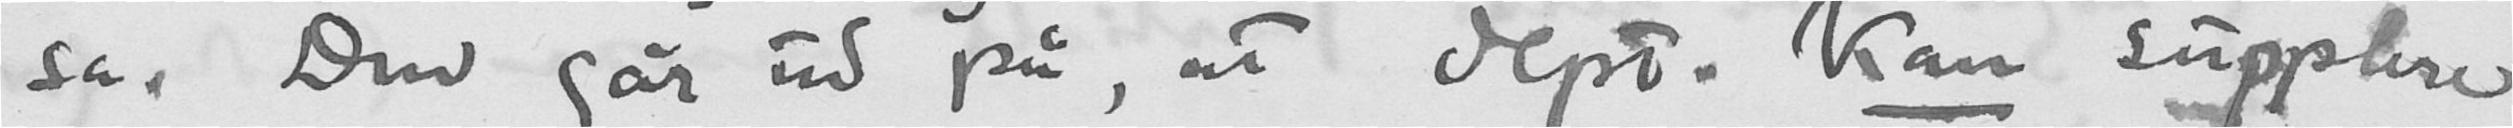sa. Den går ud på, at dept. kan supplere
